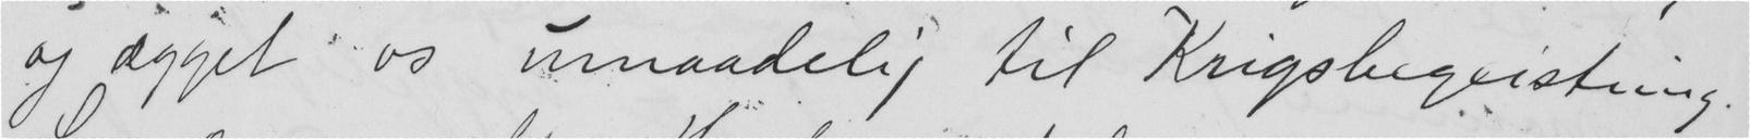og ægget os umaadelig til Krigsbegeistring.
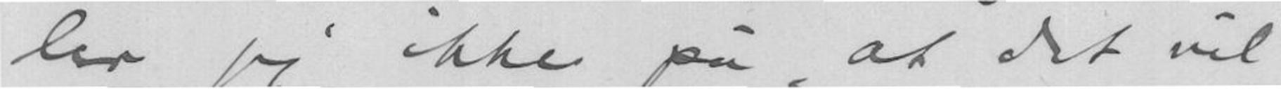ler jeg ikke på at det vil
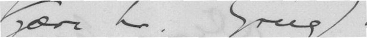Kjære hr. Grieg
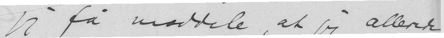jeg få meddele, at jeg allerede
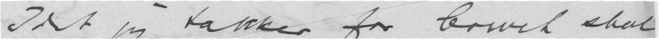Idet jeg takker for brevet skal
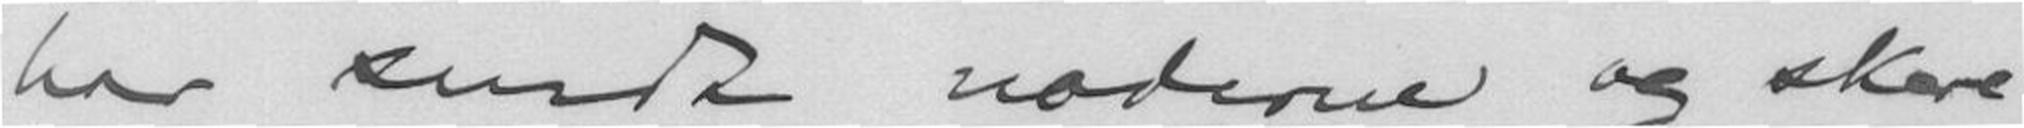har sendt noderne og skre
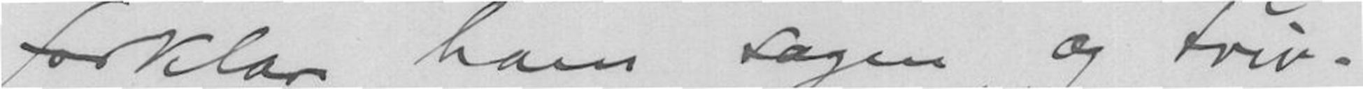forklar ham sagen, og tviv-
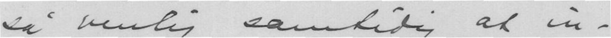så venlig samtidig at in-
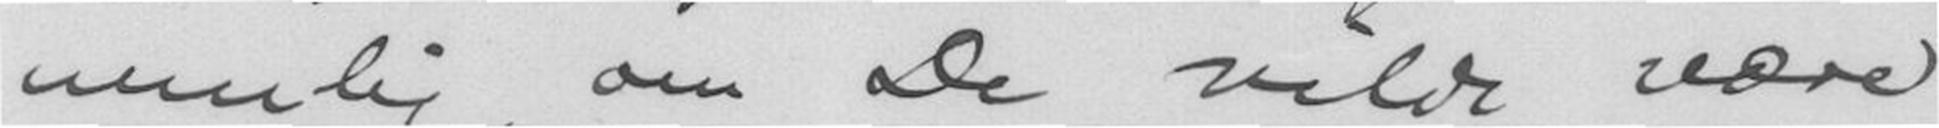nemlig, om De vilde være
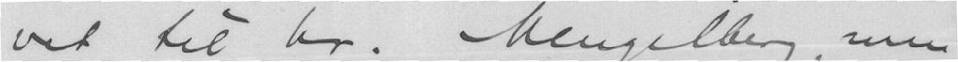vet til hr. Mengelberg, men
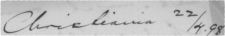Christiania 22/4.98
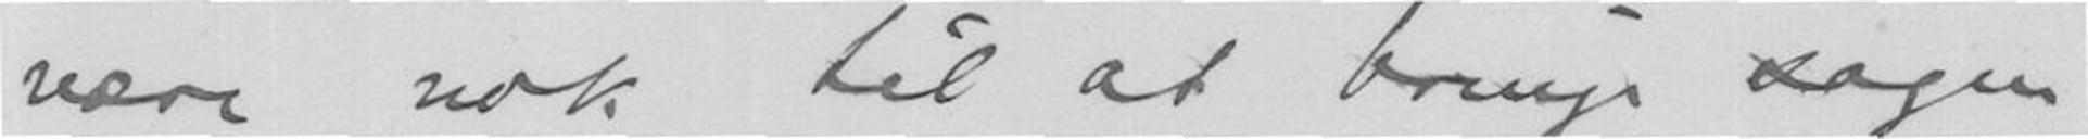være nok tid at bringe sagen
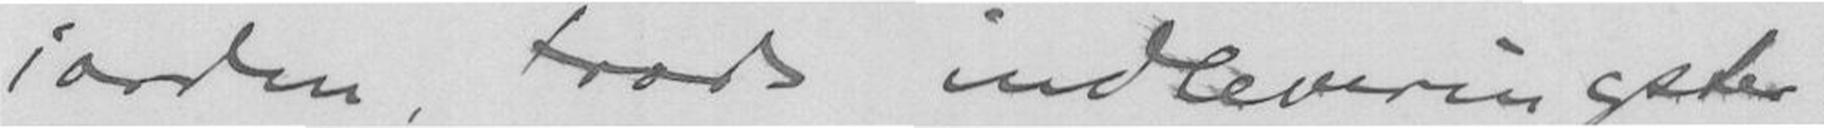iorden, trods indleveringster
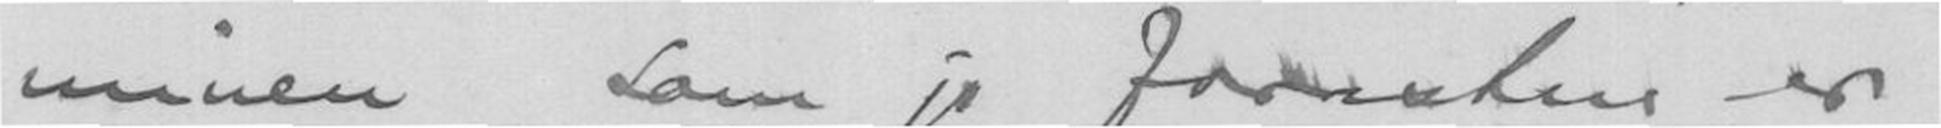minen som jo foresten er
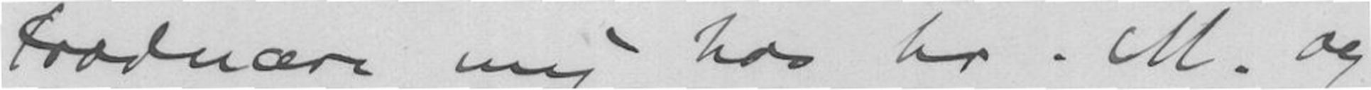troducere mig hos hr. M. og
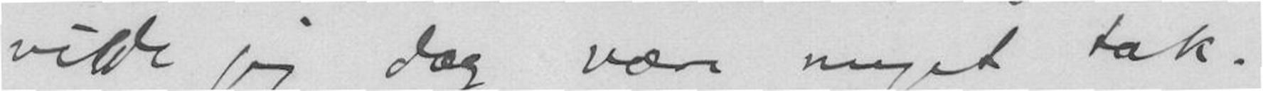vilde jeg dog være meget tak-
